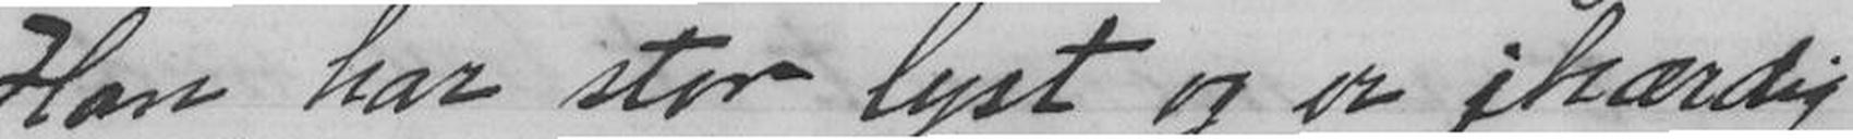Han har stor lyst og er ihærdig
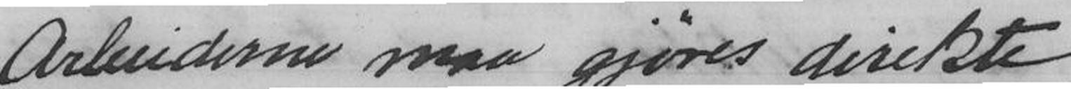Arbeiderne maa gjøres direkte
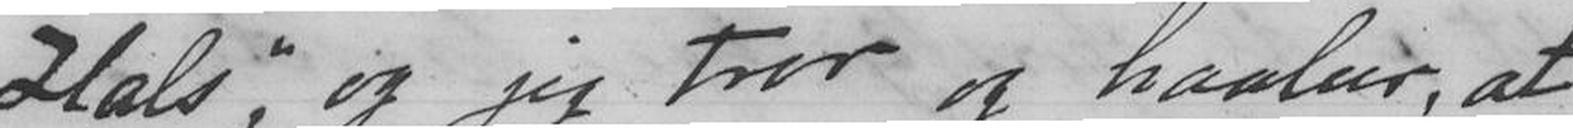Hals, og jeg tror og haaber, at
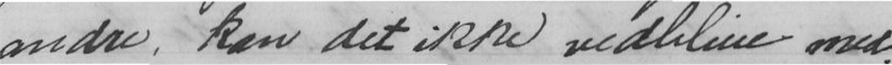andre, kan det ikke vedblive med.
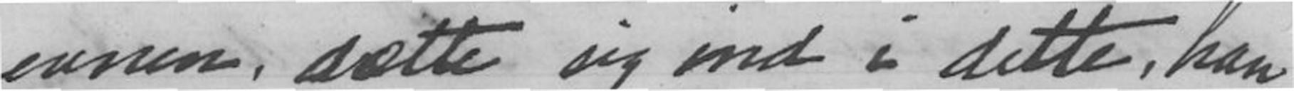evnen, sætte sig ind i dette, han
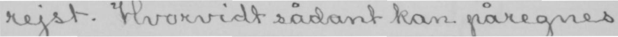rejst. Hvorvidt sådant kan påregnes
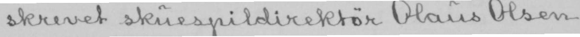skrevet skuespildirektør Olaus Olsen
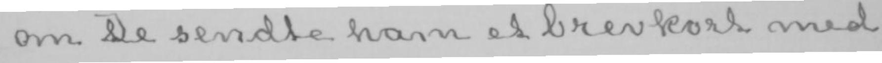om De sendte ham et brevkort med
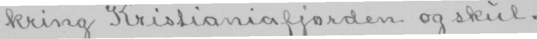kring Kristianiafjorden og skul-
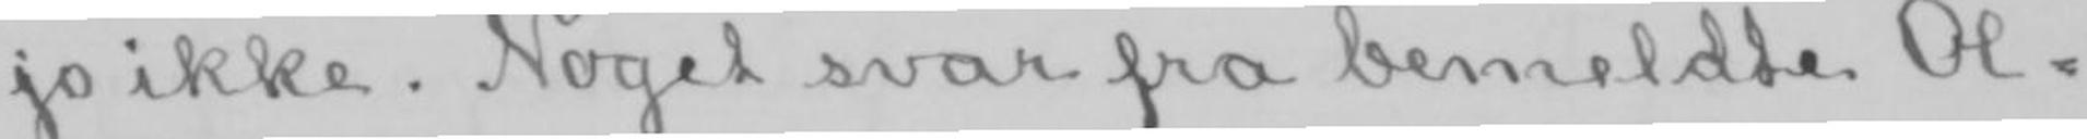jo ikke. Noget svar fra bemeldte Ol-
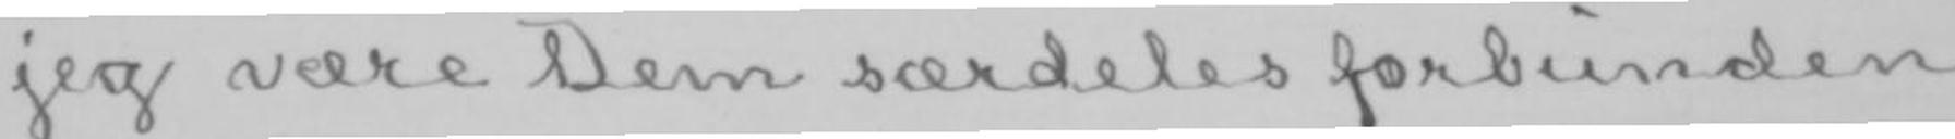jeg være Dem særdeles forbunden
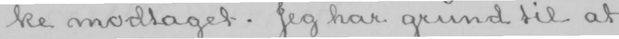ke modtaget. Jeg har grund til at
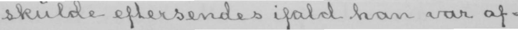skulde eftersendes ifald han var af-
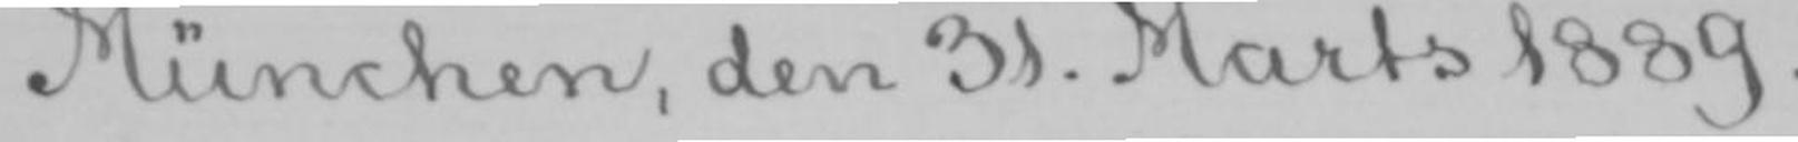München, den 31. Marts 1889.
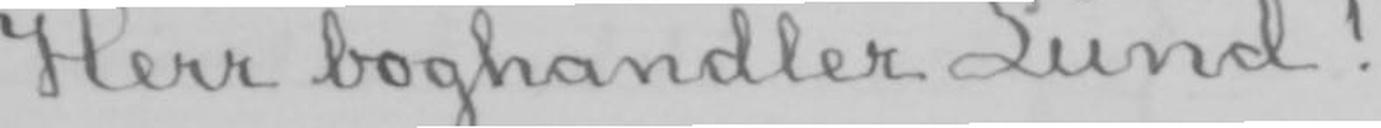Herr boghandler Lund!
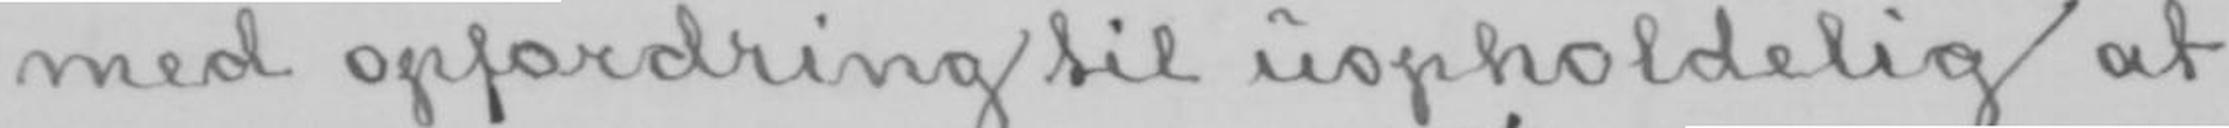med opfordring til uopholdelig at
